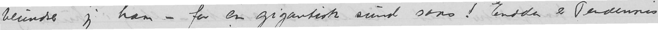beundrer jeg ham – for en gigantisk sund sans! Endda er Pendennis
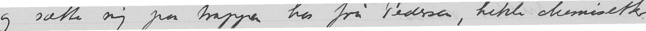og sætte mig paa trappen hos fru Pedersen, hekle chemisetter
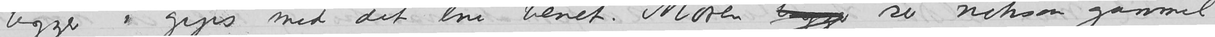ligger i gips med det ene benet. Moren bygge ser noksaa gammel
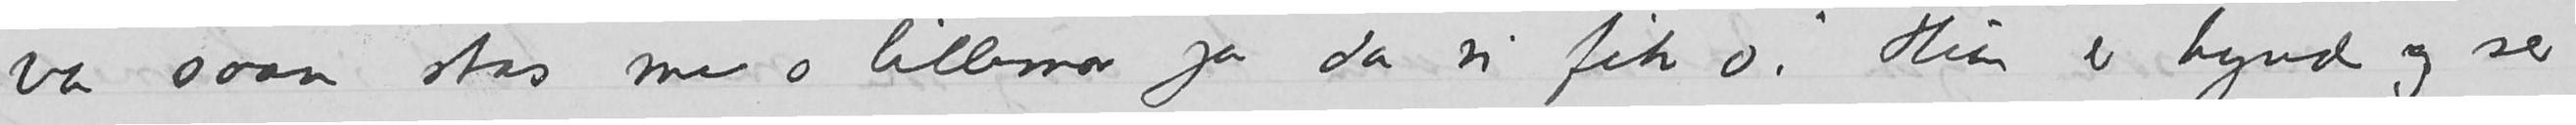var saan stas me o lillemmor ja da vi fik o.» Hun er tynd og ser
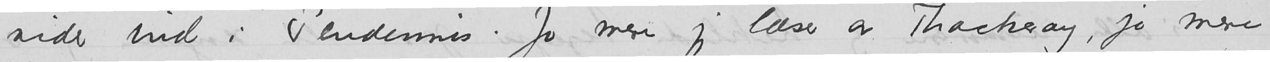sider ind i Pendennis. Jo mere jeg læser av Thackeray, jo mere
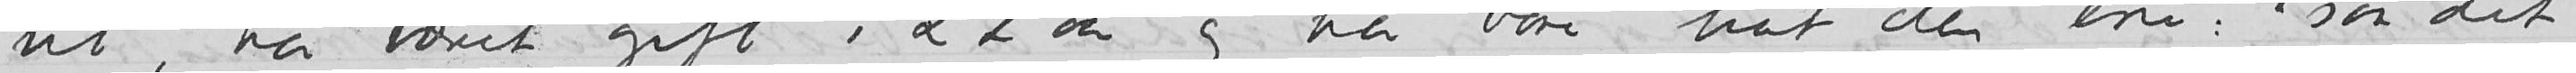ut, har været gift i 22 aar og har bare hat den ene: «saa det
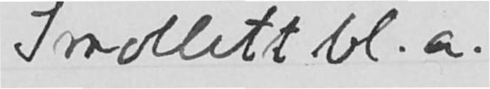Smollett bl.a.
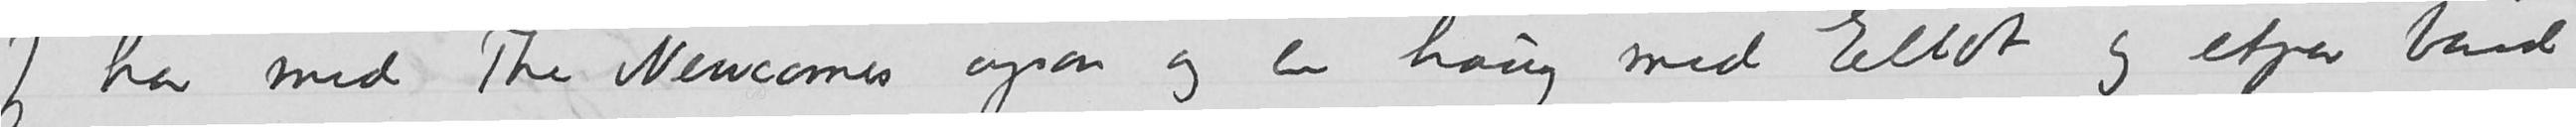Jeg har med The Newcomers ogsaa og en haug med Eliot og etpar bind
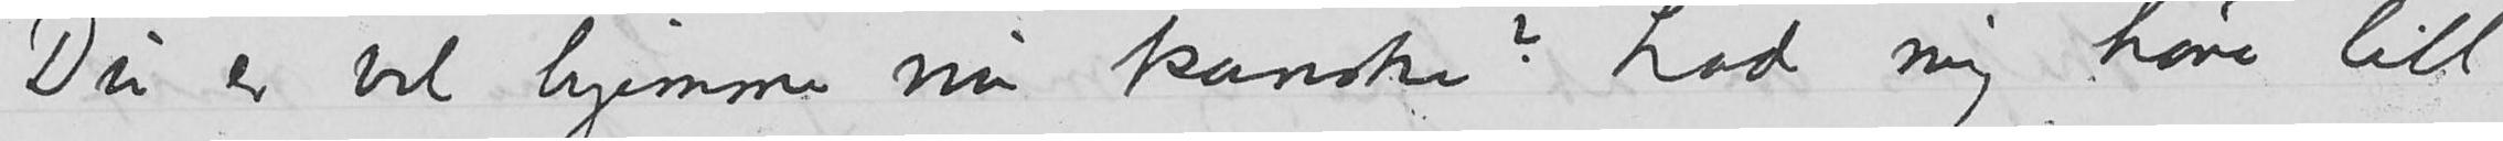Du er vel hjemme nu kanske? Lad mig høre litt
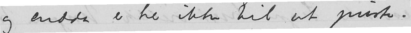og endda er her ikke til at puste.
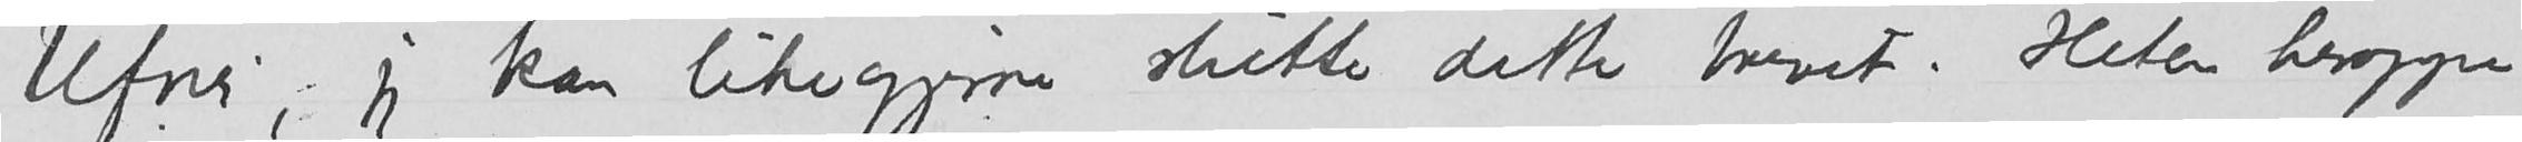Ufnei, jeg kan likegjerne slutte dette brevet. Heten heroppe
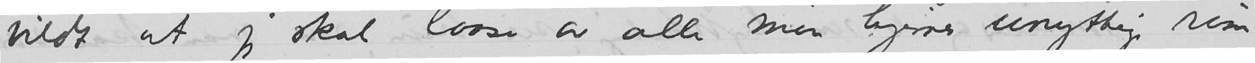vildt at jeg skal laase av alle min hjernes unyttige rum
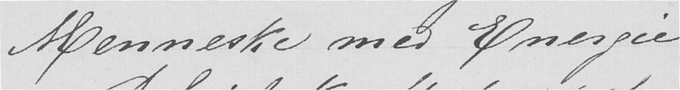Menneske med Energie
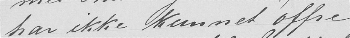har ikke kunnet offre
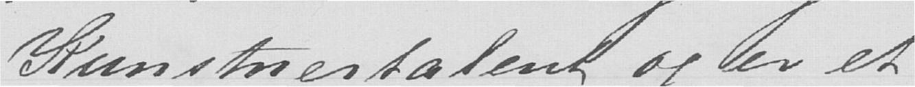Kunstnertalent og er et
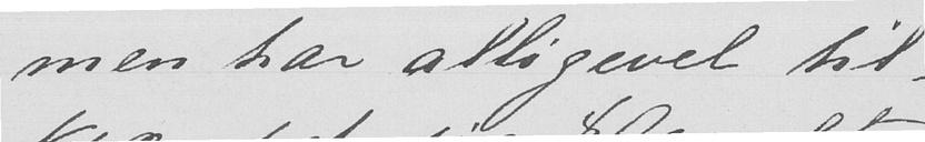men har alligevel til-
